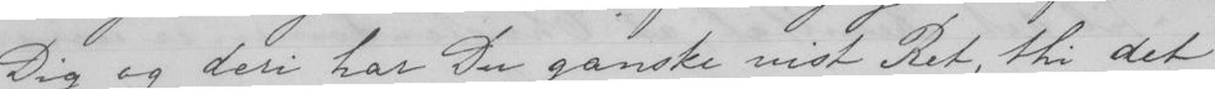Dig og deri har Du ganske vist Ret, thi det
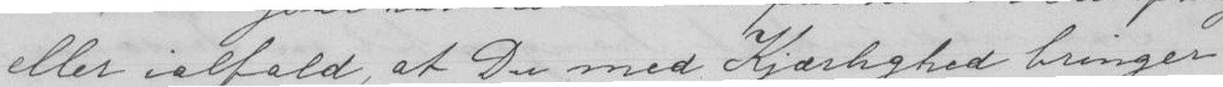eller ialfald, at Du med Kjærlighed bringer
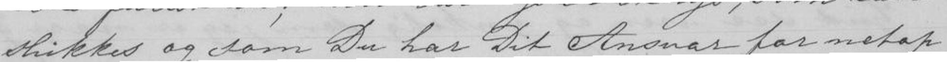skikkes og som Du har Dit Ansvar for netop
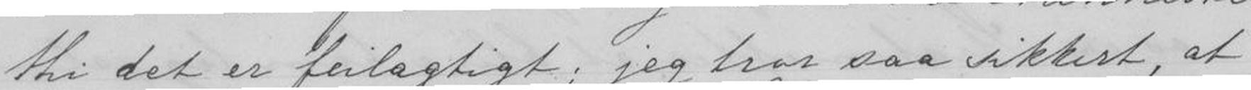thi det er feilagtigt; jeg tror saa sikkert, at
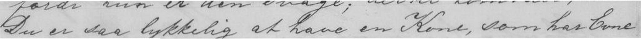Du er saa lykkelig at have en Kone, som har Evne
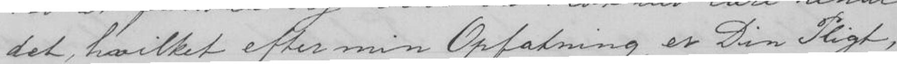det, hvilket efter min Opfatning er Din Pligt,
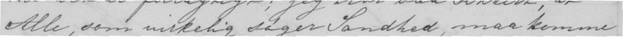Alle, som virkelig søger Sandhed, maa komme
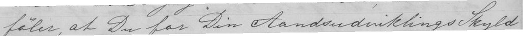føler, at Du for Din Aandsudviklings Skyld
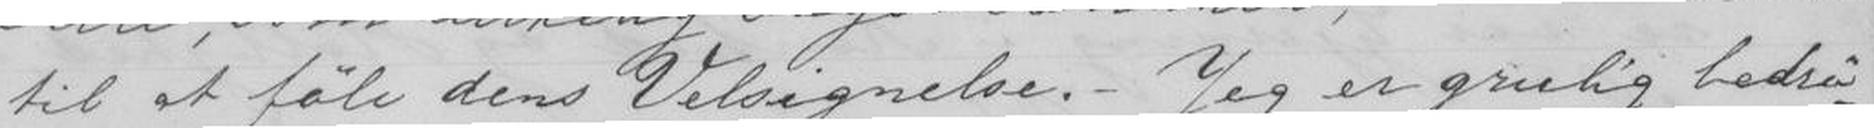til at føle dens Velsignelse.- Jeg er grulig bedrø-
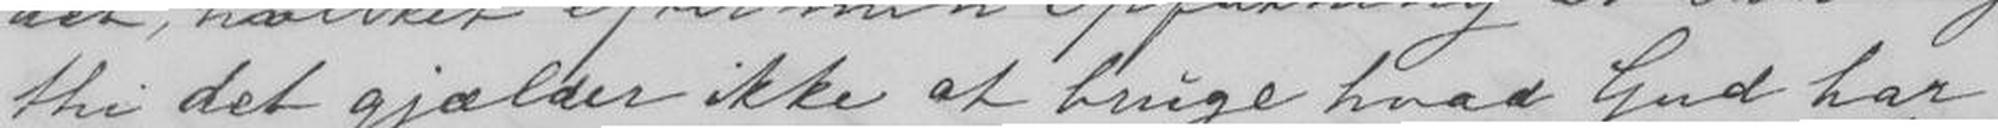thi det gjælder ikke at bruge hvad Gud har
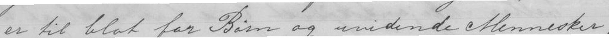er til blot for Børn og uvidende Mennesker,
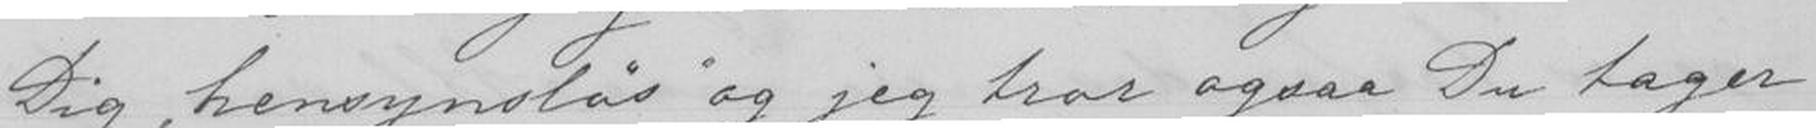Dig" hensynsløs" og jeg tror ogsaa Du tager
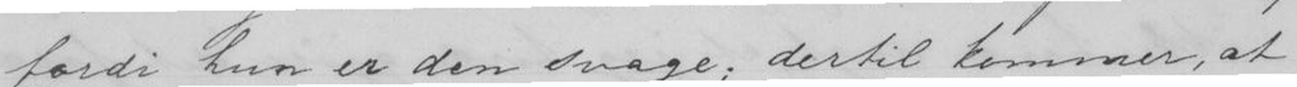fordi hun er den svage; dertil kommer, at
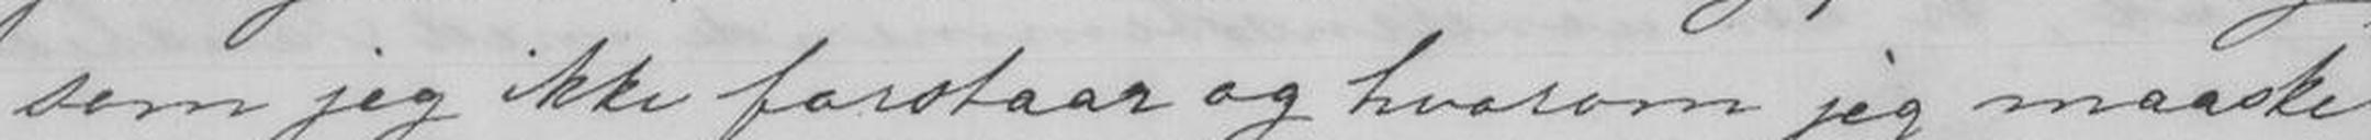som jeg ikke fostaar og hvorom jeg maaske
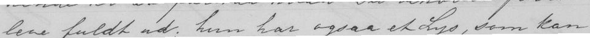leve fuldt ud; hun har ogsaa et Lys, som kan
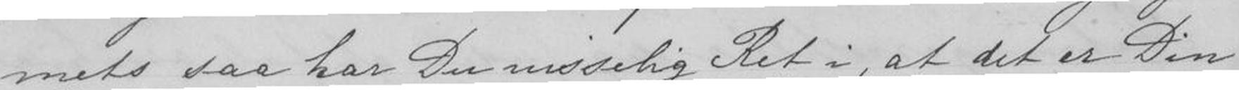mets saa har du visselig Ret i, at det er Din
